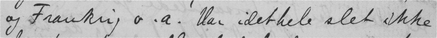og Frankrig o. a. Var idethele slet ikke
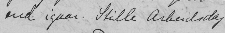end igaar. Stille arbeidsdag
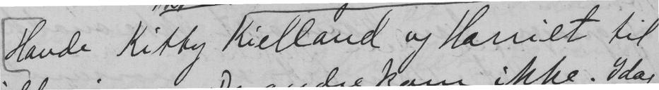Havde Kitty Kielland og Harriet til
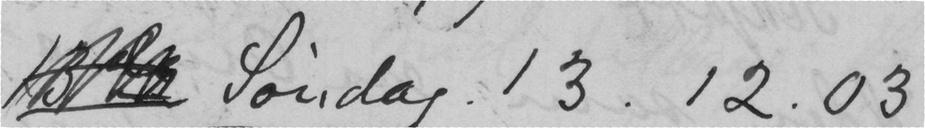Søndag 13.12.03
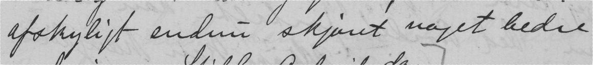afskyligt endnu skjønt noget bedre
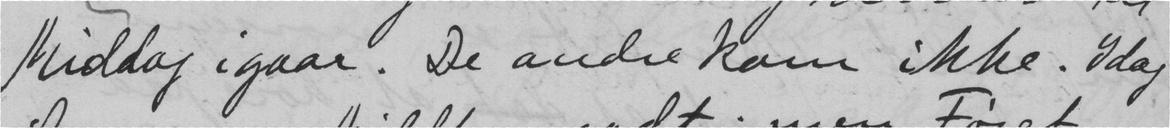middag igaar. De andre kom ikke. Idag
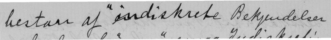bestaar af "indiskrete Bekjendelser
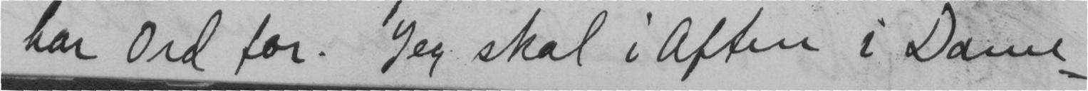har Ord for. Jeg skal i aften i Dame-
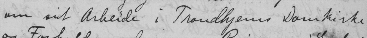om sit arbeide i Trondhjems Domkirke
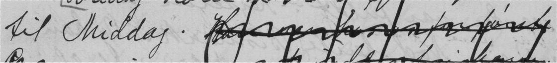til middag.
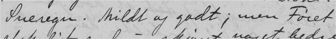Sneregn. Mildt og godt; men Føret
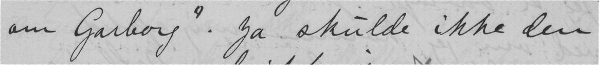om Garborg". Ja skulde ikke den
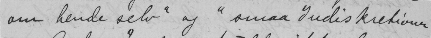om hende selv" og" smaa Indiskretioner
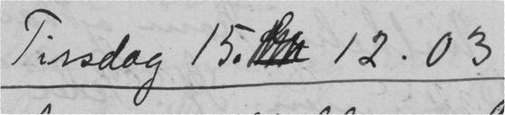Tirsdag 15. 12.03
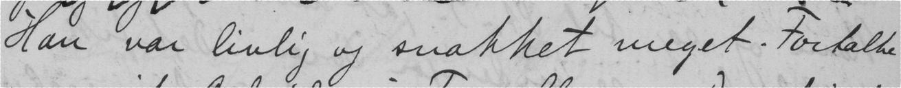Han var livlig og snakket meget. Fortalte
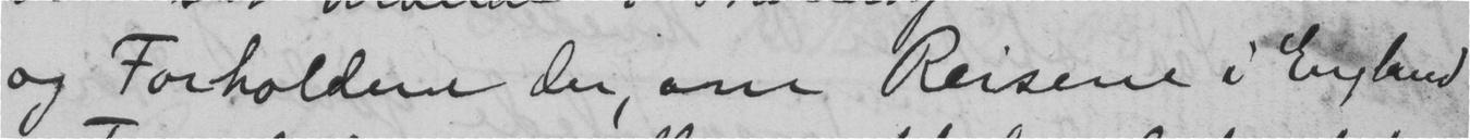og Forholdene der, om Reisene i England
